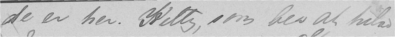de er her. Kitty, som ber at hilse
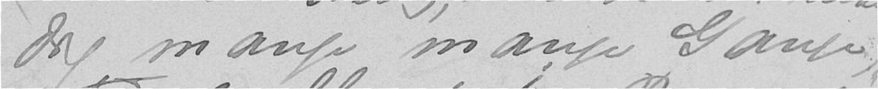dig mange mange Gange,
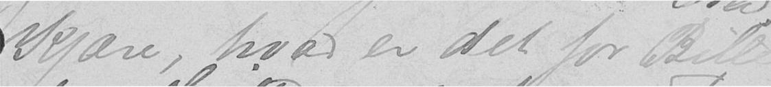Kjære, hvad er det for Bille-
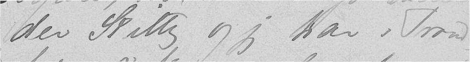der Kitty og jeg har i Trond-
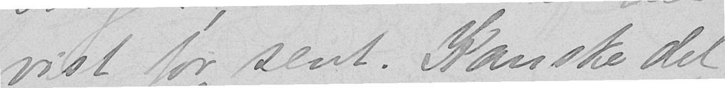vist for sent. Kanske det
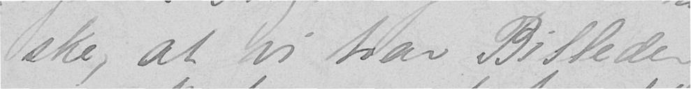ske, at vi har Billeder
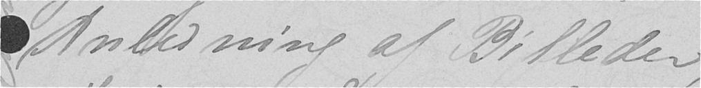Anledning af Billeder,
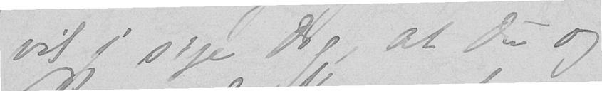vil jeg sige dig, at du og
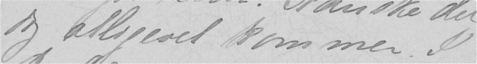dog alligevel kommer. I
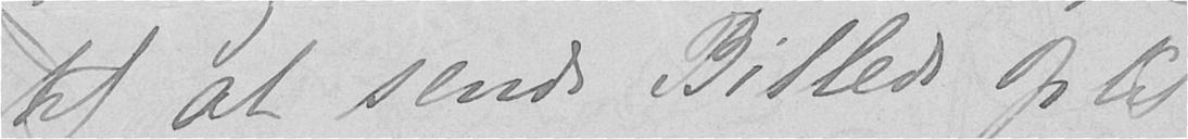til at sende Billede op til
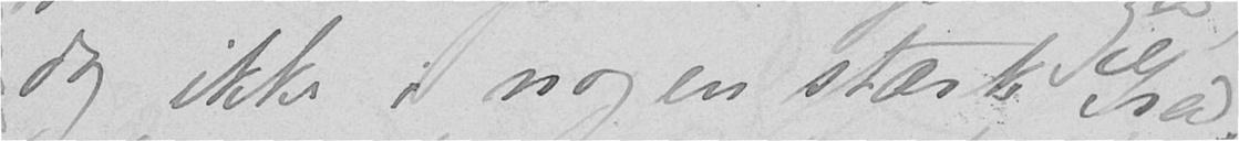dog ikke i nogen stærk Grad.
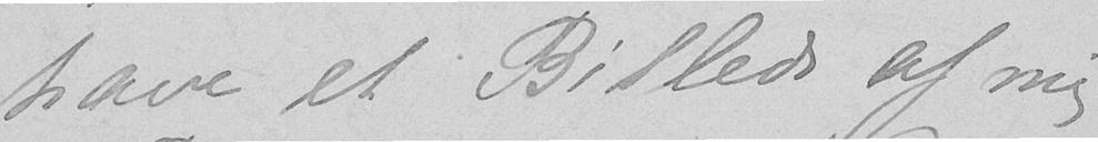have et Billede af mig
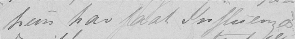hun har faat Influenza,
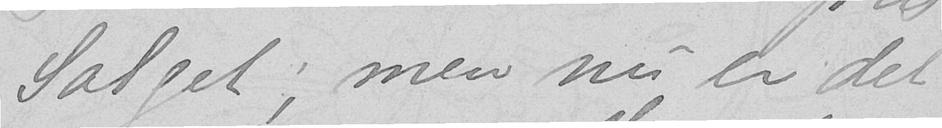Salget; men nu er det
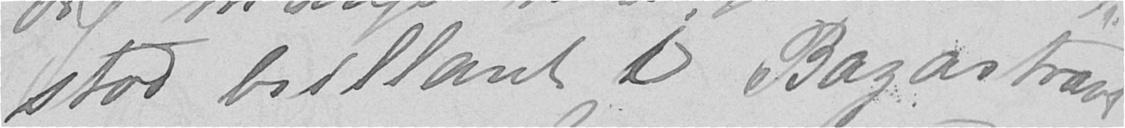stod brillant i Bazartravl-
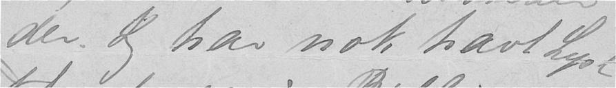der. Jeg har nok havt Lyst
